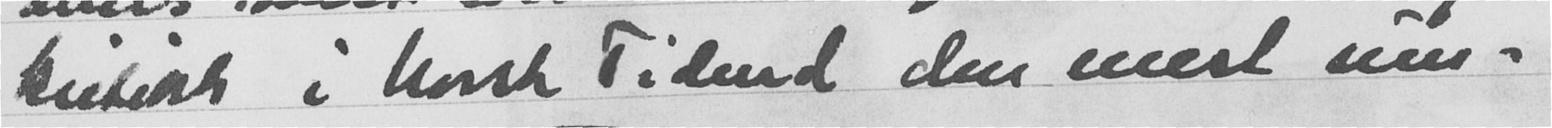kritikk i Norsk Tidend den mest um-
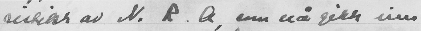risterik av N. R. A, som nå gikk inn
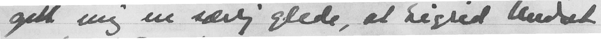gitt mig en særlig glede, at Sigrid Undset
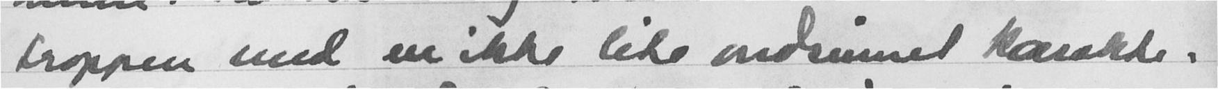troppen med en ikke lite ondsinnet karakte-
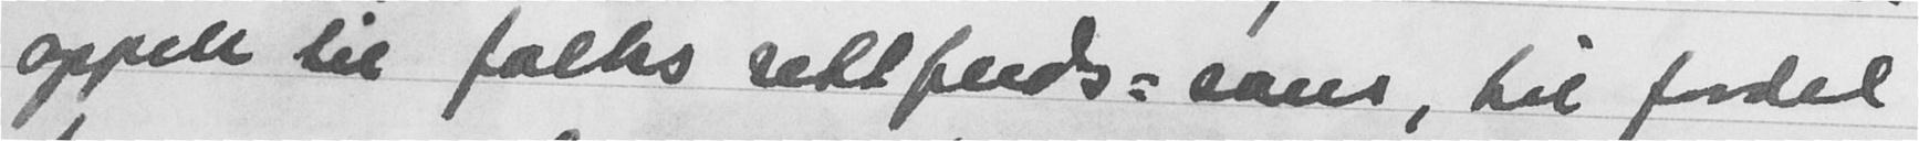appell til folks rettferds-sans, til fordel
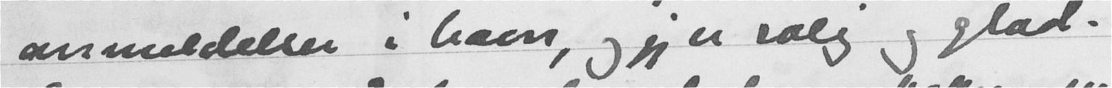anmeldelser i havn, og jeg er salig og glad.
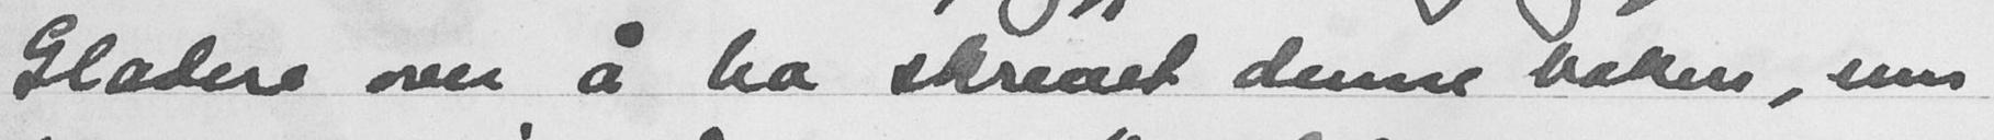Gladere over å ha skrevet denne boken, enn
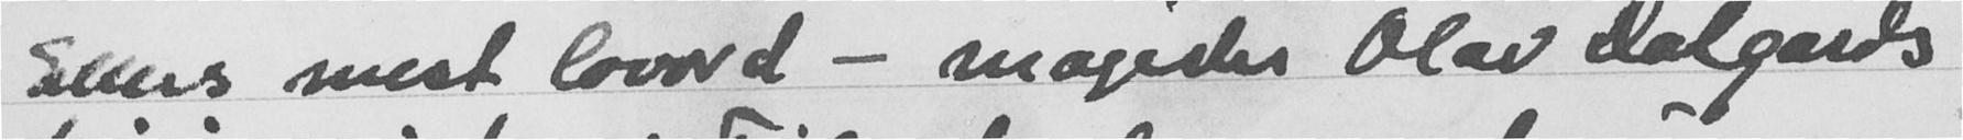Ellers mest lovord - magister Olav Dalgards
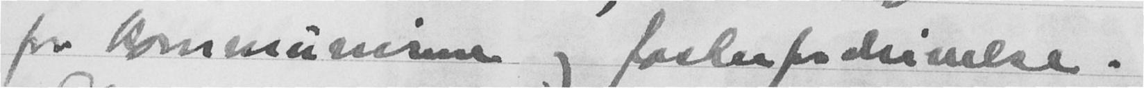for kommunisme og fosterfordrinelse.
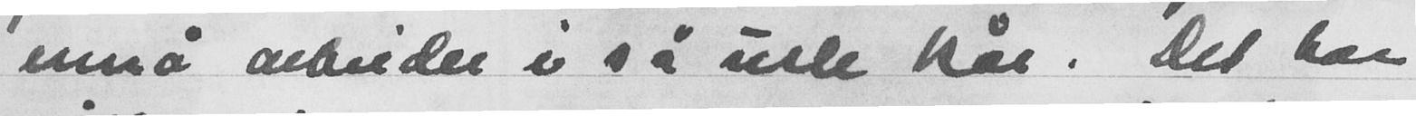ennå arbeider i så usle kår. Det har
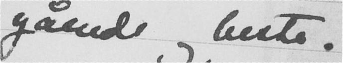gående og beste.
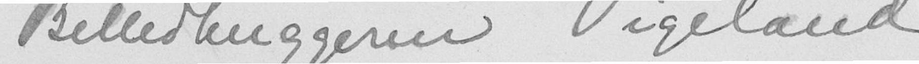Billedhuggeren Vigeland
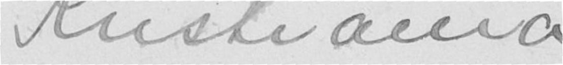Kristiania
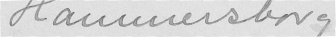Hammersborg
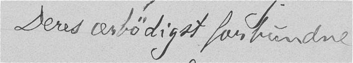Deres ærbødigst forbundne
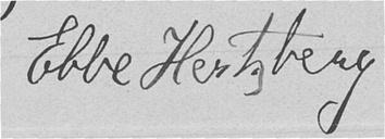Ebbe Hertzberg
